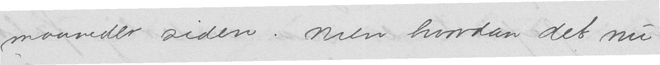maaneder siden. Men hvordan det nu
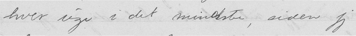hver uge i det mindste, siden jeg
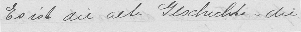Es ist die alte Geschichte - die
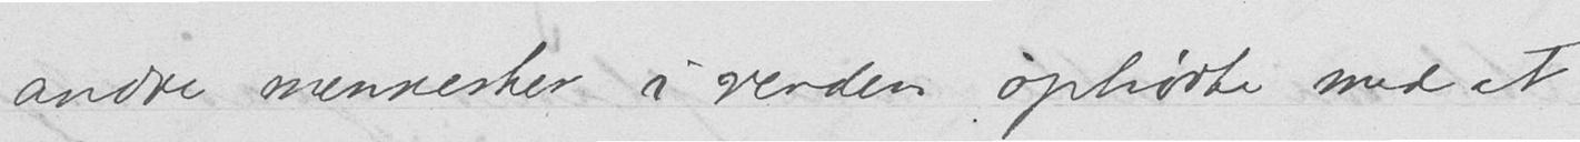andre mennesker i verden ophørte med at
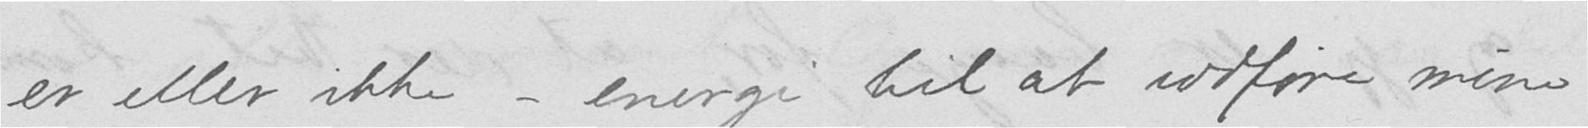er eller ikke - energi til at udføre mine
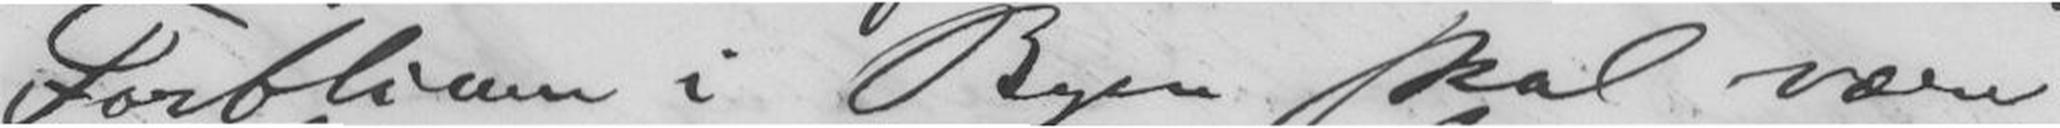Forbliven i Byen skal være
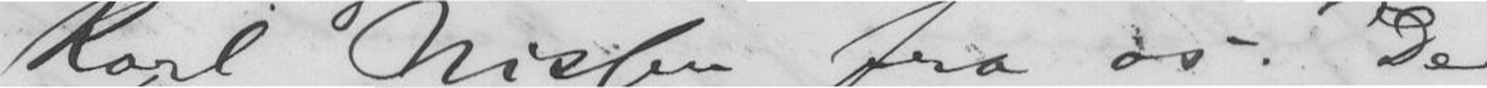Karl Nissen fra os. De
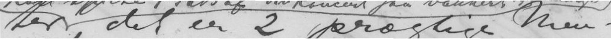ter, det er 2 prægtige Men-
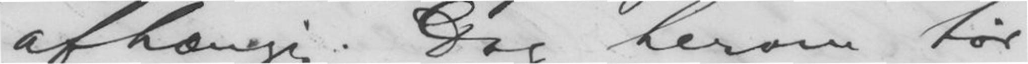afhængi. Dog herom tør
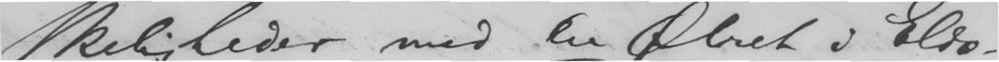skeligheder med en Ølret i Eldo-
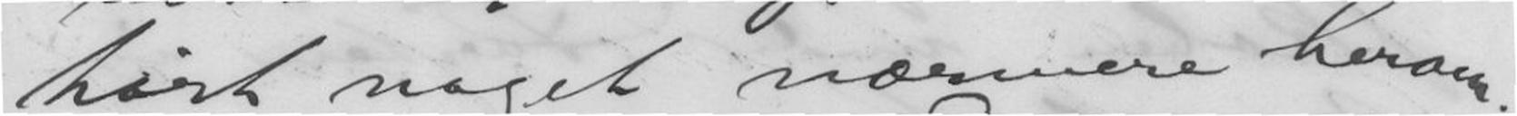hørt noget nærmere herom.
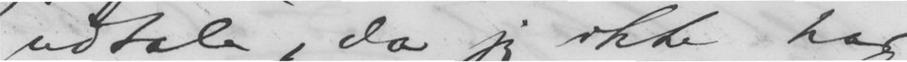udtale, da jeg ikke har
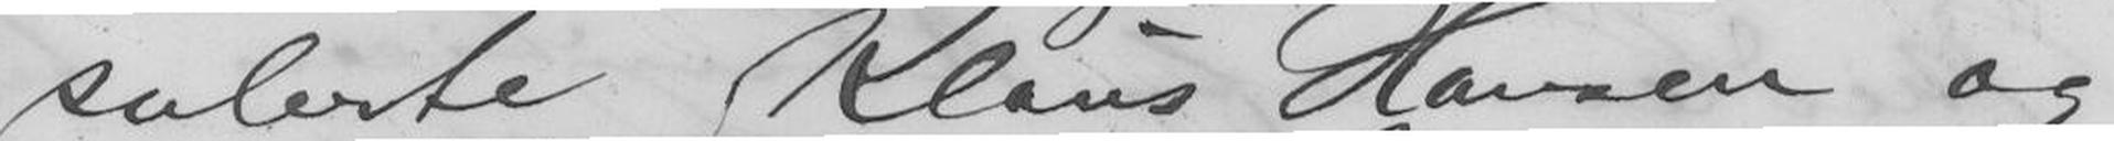sulerte Klaus Hansen og
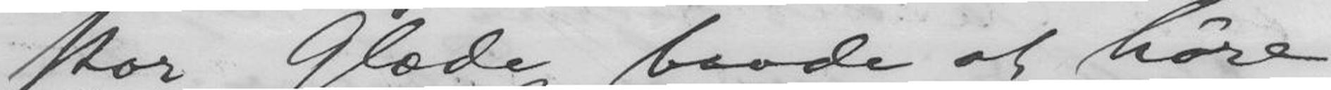stor Glæde baade at høre
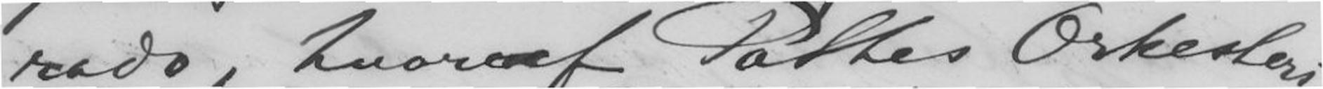rado, hvoraf Pathes Orkesters
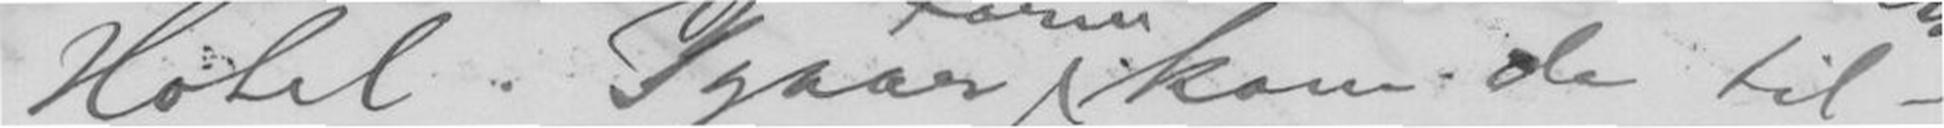Hotel. kom Ole til-
